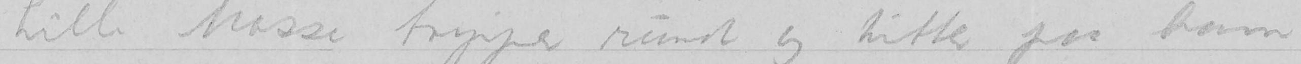Lille Mosse tripper rundt og titter paa ham
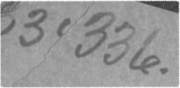336.
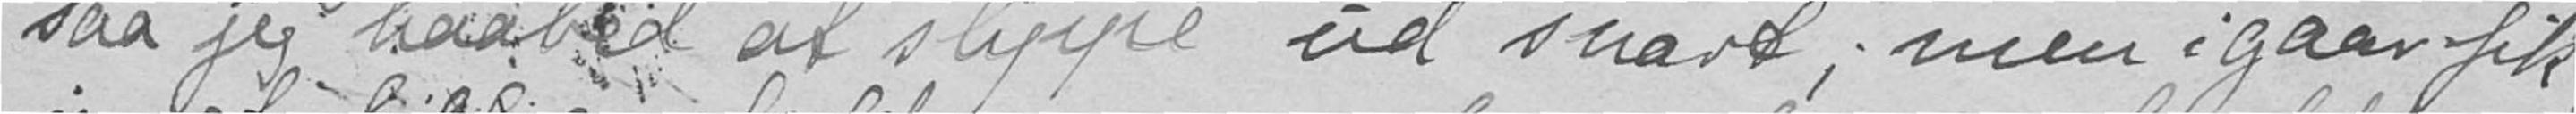saa jeg haabed at slippe ud snart, men igaar fik
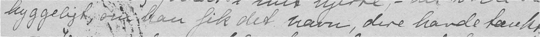hyggeligt, om han fik det navn dere havde tænkt.
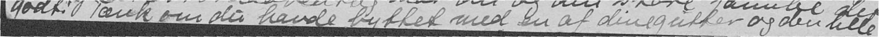godt. Tænk, om du havde byttet med en af dine gutter og den lille
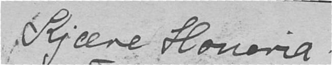Kjære Honoria!
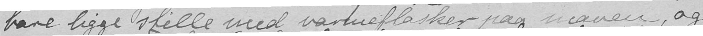bare ligge stelle med varmeflasker paa maven, og
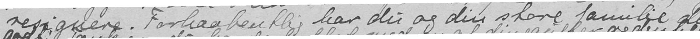resignere. Forhaabentlig har du og din store familie det
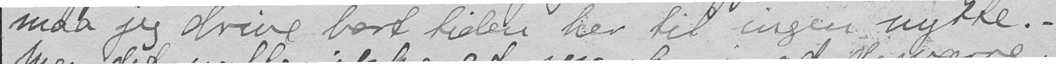maa jeg drive bort tiden her til ingen nytte.-
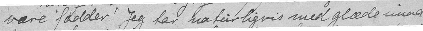være fadder! Jeg tar naturligvis med glæde imod
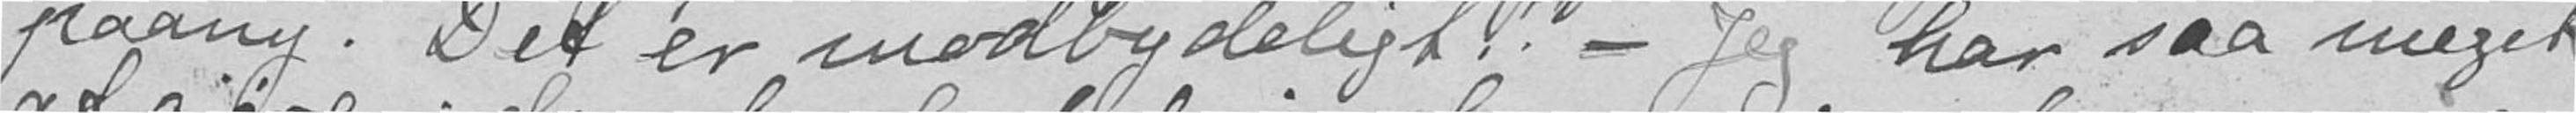paany. Det er modbydeligt. - Jeg har saa meget
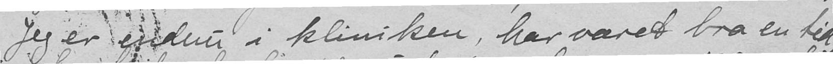- Jeg er endnu i kliniken, har været bra en tid
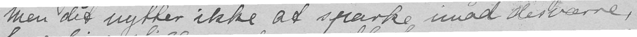Men det nytter ikke at sparke imod desværre,
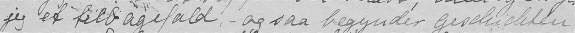jeg et tilbagefald,- og saa begynder gesdvideten
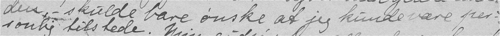den,- skulde bare ønske at jeg kunde være per-
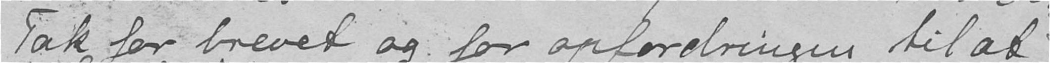Tak for brevet og for opfordringen til at
